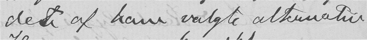det af ham valgte alternativ.
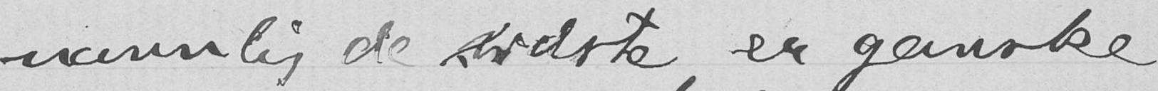navnlig de sidste, er ganske
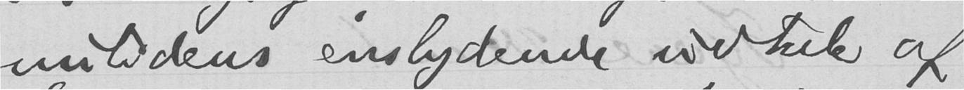nutidens enslydende udtale af
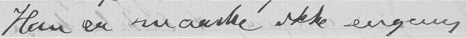Han er maaske ikke engang
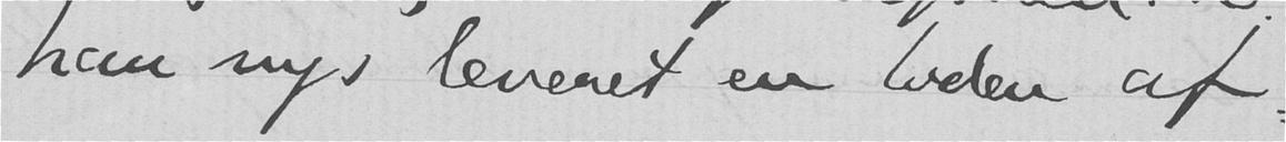har nys leveret en liden af-
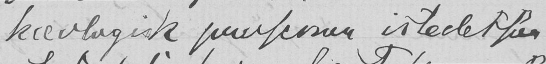kæologisk professor istedetfor
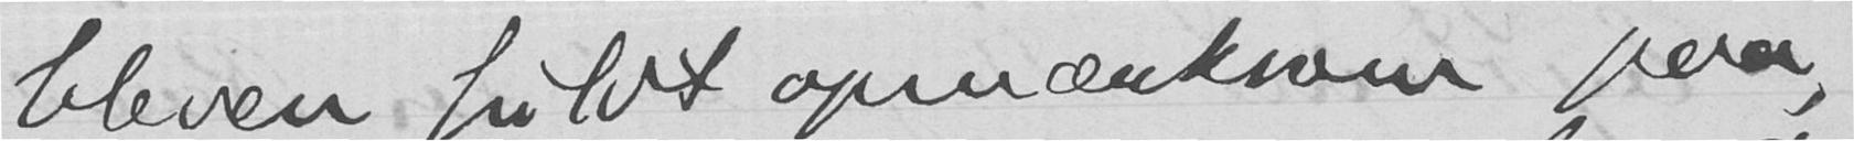bleven fuldt opmærksom paa
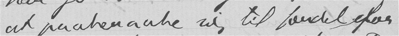at paaberaabe sig til fordel for
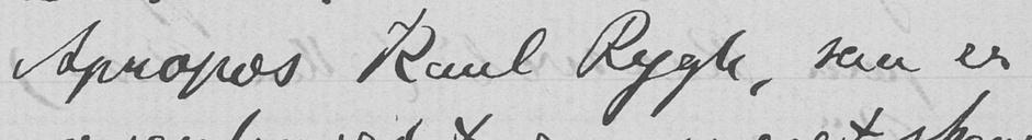Apropos Karl Rygh, saa er
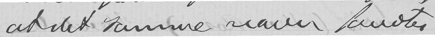at det samme navn fandtes
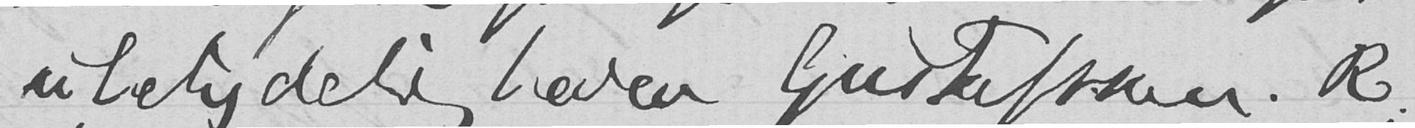ubetydeligheden Gustafsson. R.
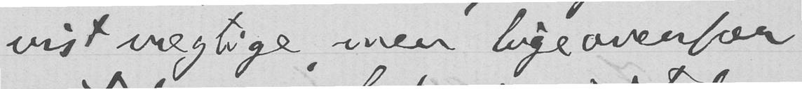vist vægtige, men ligeoverfor
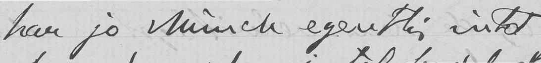har jo Munch egentlig intet
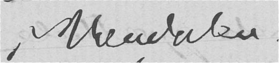i Neadalen.
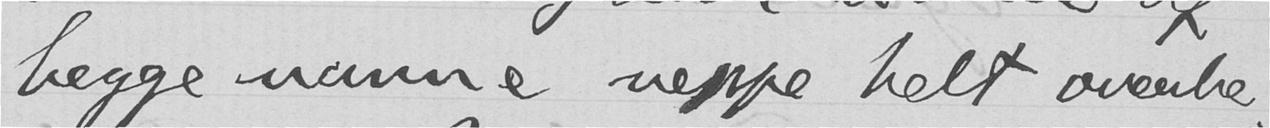begge navne neppe helt overbe-
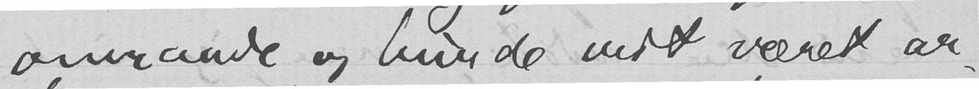omraade og burde vist været ar-
